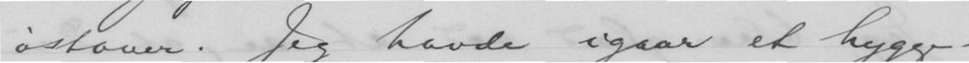østover. Jeg havde igaar et hygge-
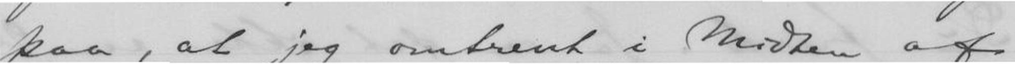paa, at jeg omtrent i Midten af
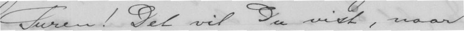Turen! Det vil Du vist, naar
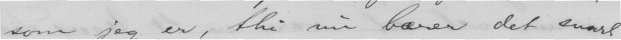som jeg er, thi nu bærer det snart
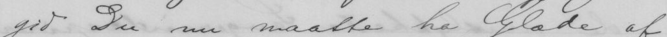gid Du nu maatte ha Glæde af
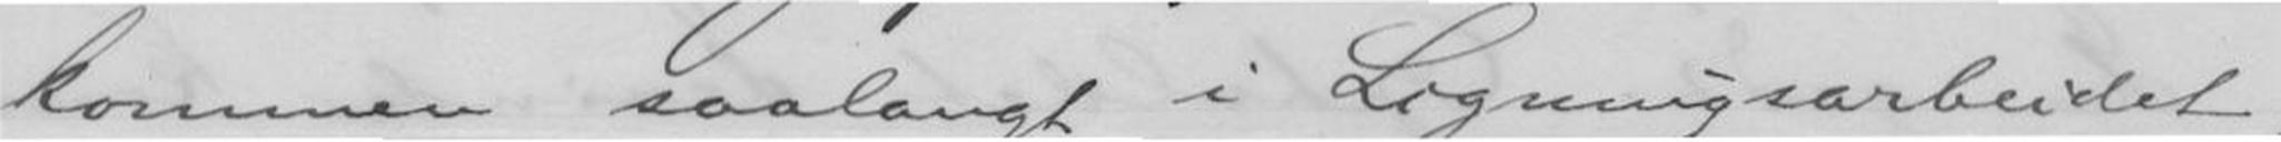kommen saalangt i Ligningsarbeidet,
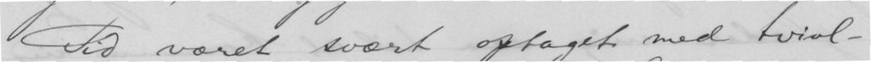Tid været svært optaget med tvivl-
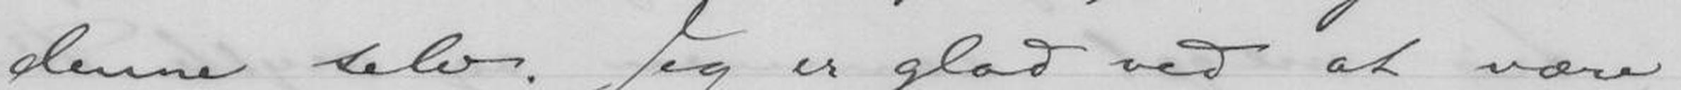denne selv. Jeg er glad ved at være
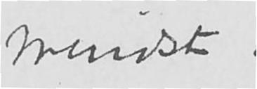mindst.
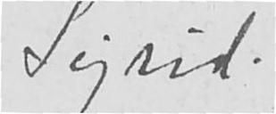Sigrid
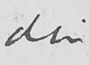din
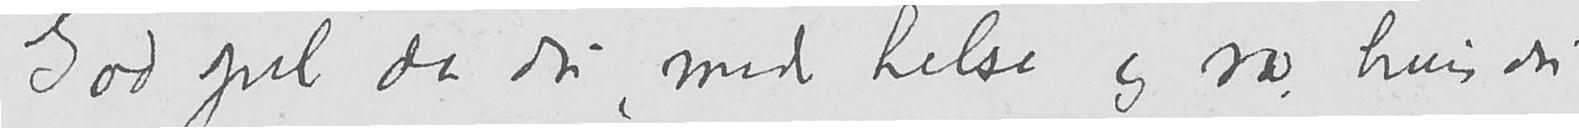God jul da du, med helse og ro hvis du
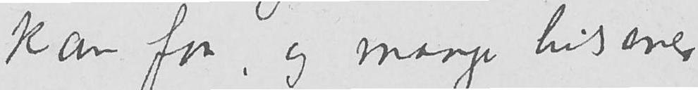kan faa, og mange hilsener
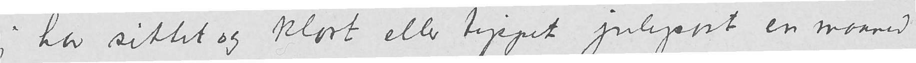Jeg har sittet og klort eller tippet julepost en maaned.
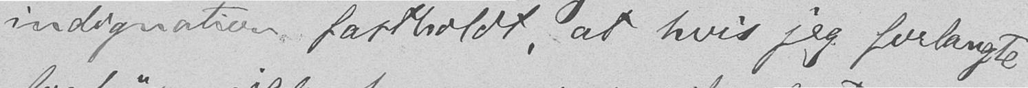indignation fastholdt, at hvis jeg forlangte
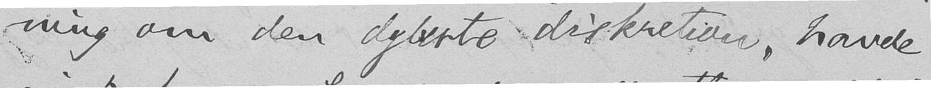ning om den dybeste diskretion, havde
